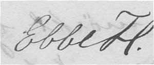Ebbe H.
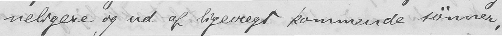neligere og ud af ligevægt kommende sønner,
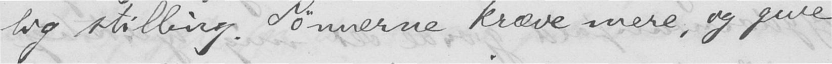lig stilling. Sønnerne kræve mere, og give
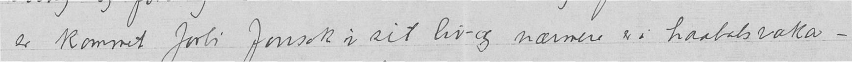er kommet forbi Jonsok i sit liv og nærmere er i haabalsvaka – .
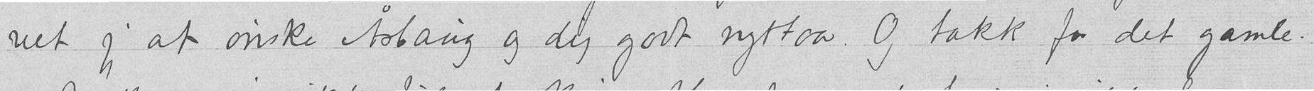vet jeg at ønske Åslaug og dig godt nyttaar. Og takk for det gamle.
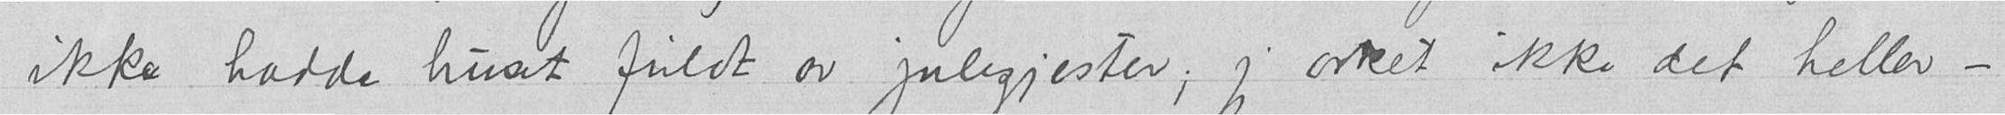ikke hadde huset fuldt av julegjester; jeg orket ikke det heller –
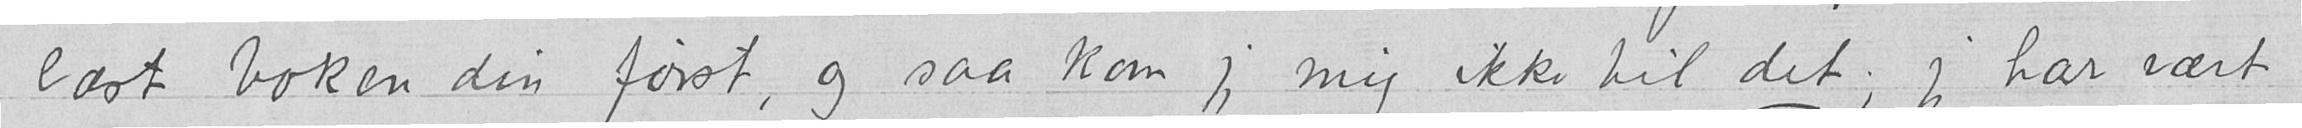læst boken din først, og saa kom jeg mig ikke til det; jeg har vært
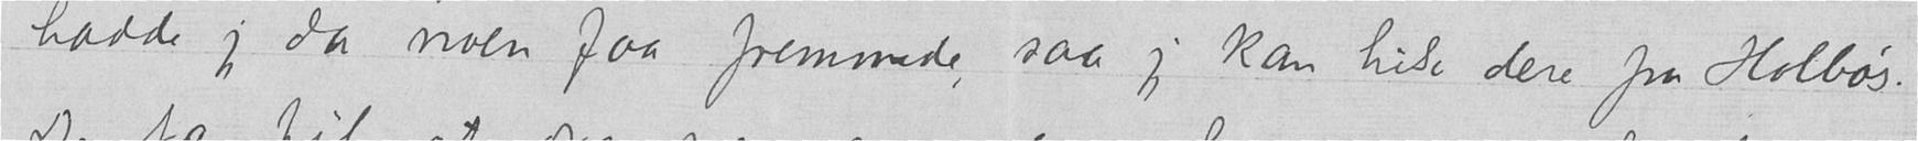hadde jeg da noen faa fremmede, saa jeg kan hilse dere fra Holbøs.
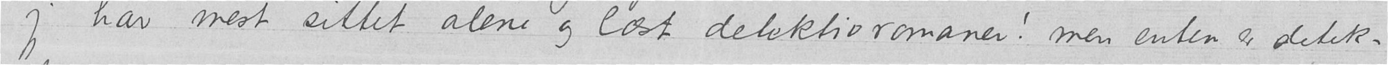jeg har mest sittet alene og læst detektivromaner! men enten er detek-
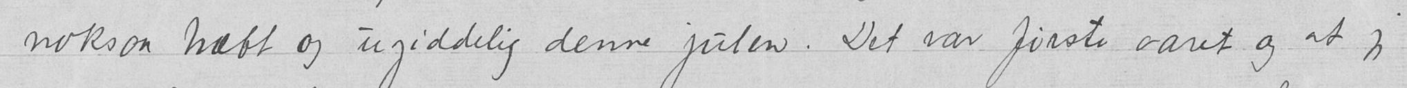noksaa trætt og ugiddelig denne julen. Det var første aaret og at jeg
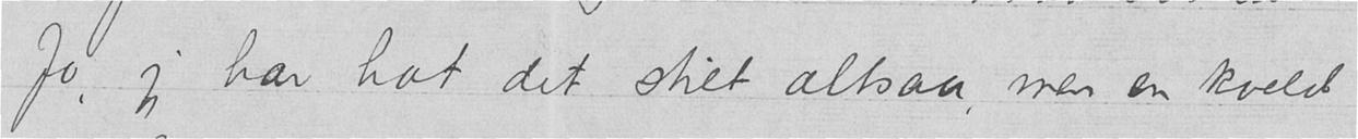Jo, jeg har hat det stilt altsaa, men en kveld
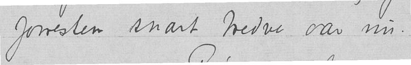forresten snart tredve aar nu.
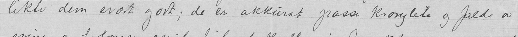likte dem svært godt; de er akkurat passe kronglete og fulde av
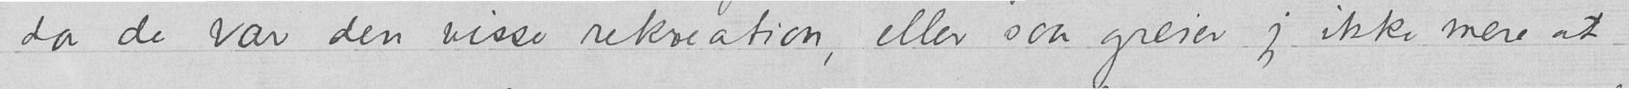da de var den visse rekreation, eller saa greier jeg ikke mere at
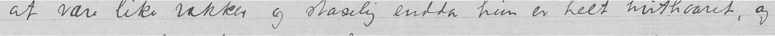at være like vakker og staselig endda hun er helt hvithaaret, og
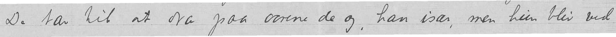De tar til at dra paa aarene de og, han især, men hun blir ved
